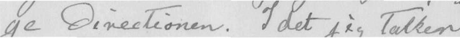ge Directionen. I det jeg Takker
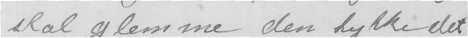skal glemme den lykke det
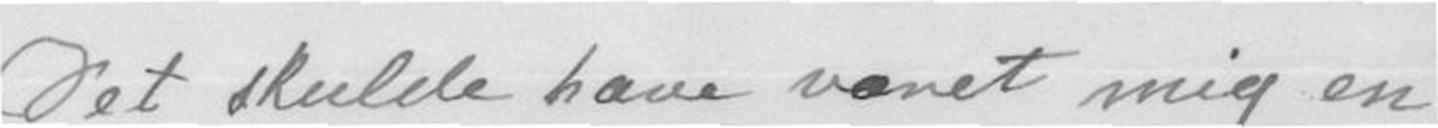Det skulde have været mig en
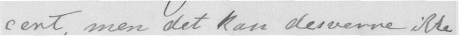cert, men det kan desverre ikke
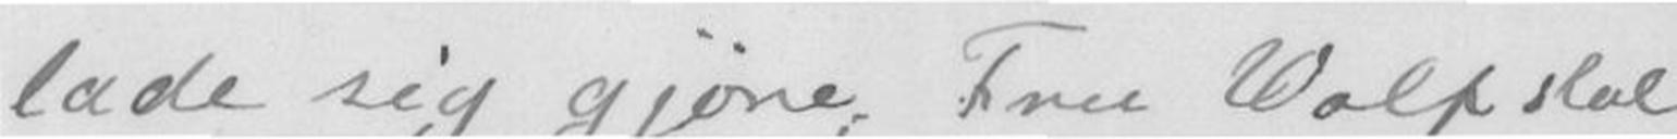lade sig gjøre; Fru Wolfstal
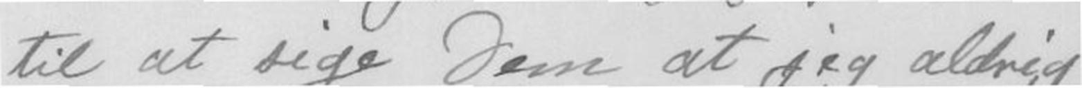til at sige Dem, at jeg aldrig
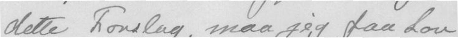dette Forslag, maa jeg faa Lov
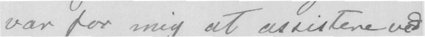var for mig at assistere ved
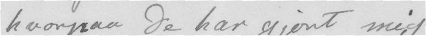hvorpaa De har gjort mig
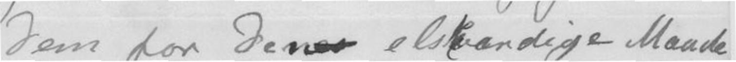Dem for Deres elskværdige Maade
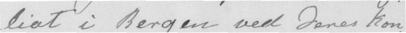liot i Bergen ved Deres Kon-
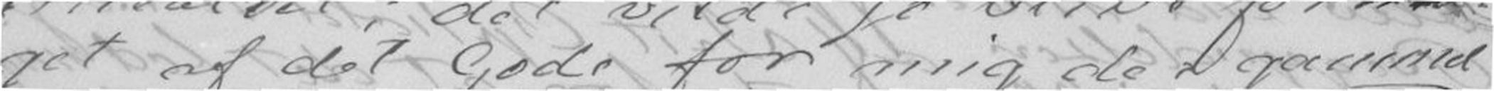get af det gode for mig der gammel
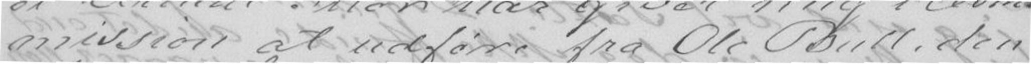mission at udføre fra Ole Bull, den
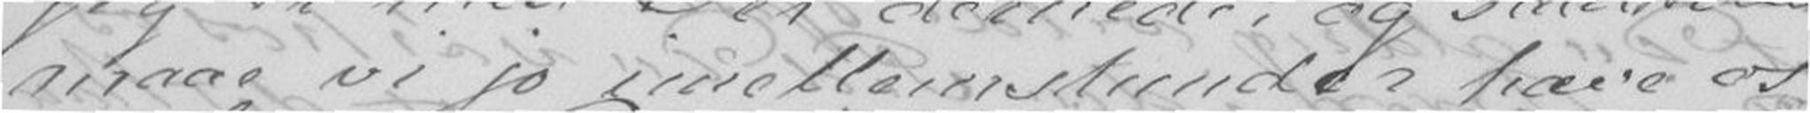maae vi jo imellemstunder have os
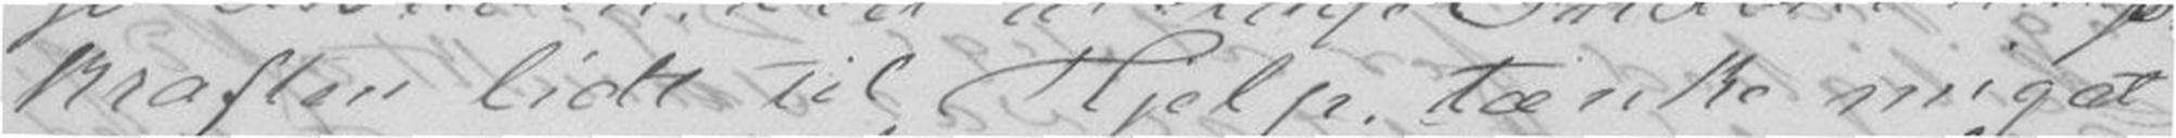kraften lidt til Hjelp, tænke mig at
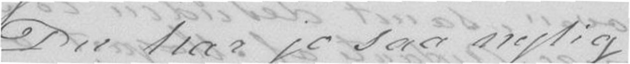Du har jo saa nylig
